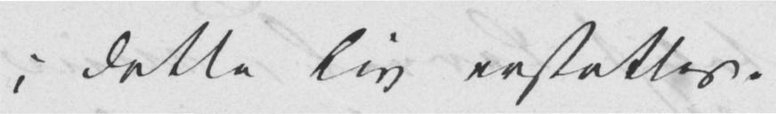i dette Liv erstattes.
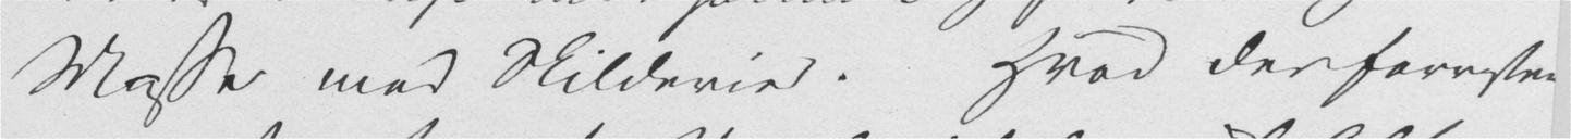Masse med Skilderier. Hvad der forresten
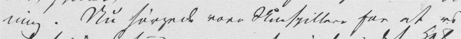ning. Nu sørgede vore Skuespillere for at vi
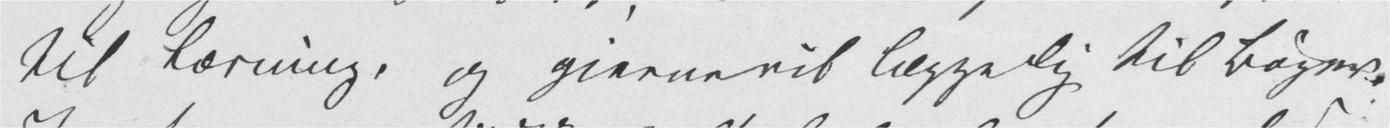til Læsning, og gjerne vil lægge Dig til Bøger.
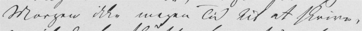Morgen ikke megen Tid til at skrive,
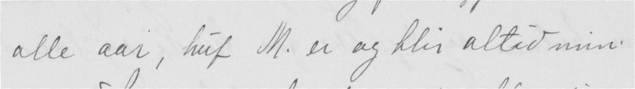alle aar, huf M. er og bli altid min
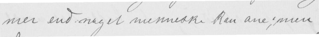mer end noget menneske kan ane; men
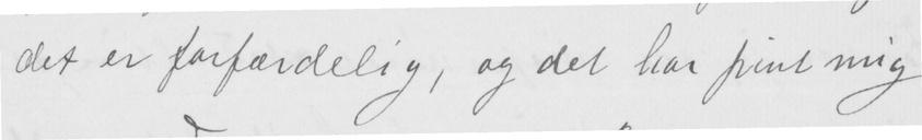det er forfærdelig, og det har pint mig
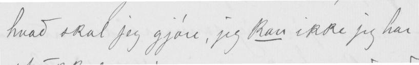hvad skal jeg gjøre, jeg kan ikke jeg har
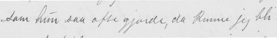som hun saa ofte gjorde, da kunne jeg bli
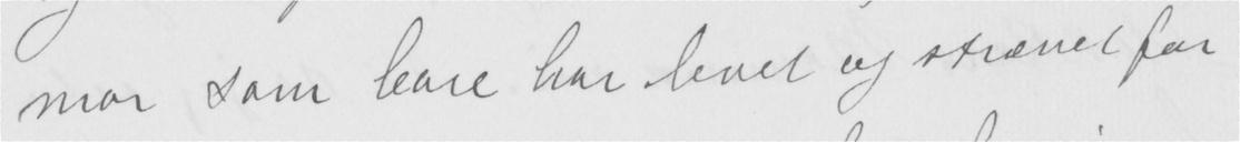mor som bare har levet og strævet for
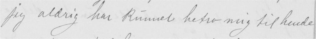jeg aldrig har kunnet betro mig til hende
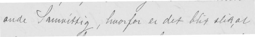onde Samvittig, hvorfor er det blit slik, at
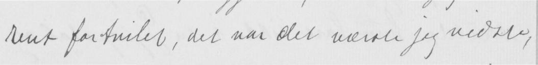rent fortvilet, det var det værste jeg vidste,
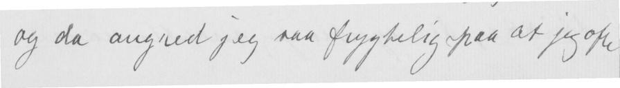og da angred jeg saa frygtelig paa at jeg ofte
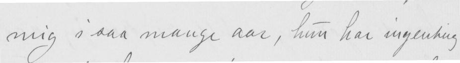mig i saa mange aar, hun har ingenting
